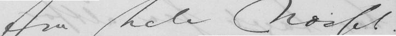fra hele Næsset.
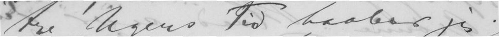tre Ugers Tid haaber jeg.
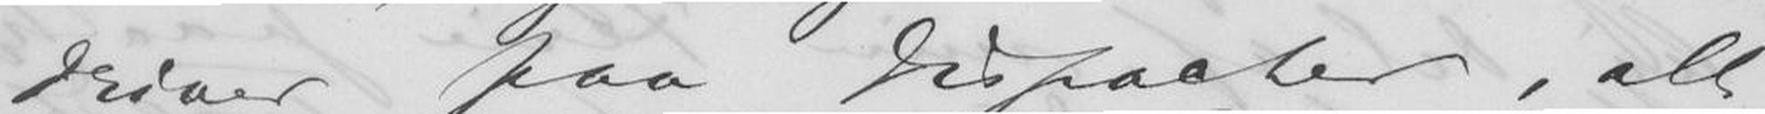driver paa Dispacher, alt
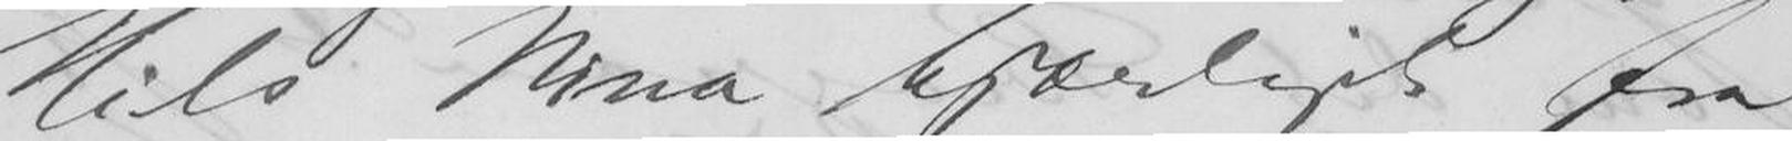Hils Nina kjærligt fra
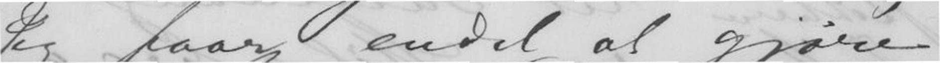Jeg faar endel at gjøre
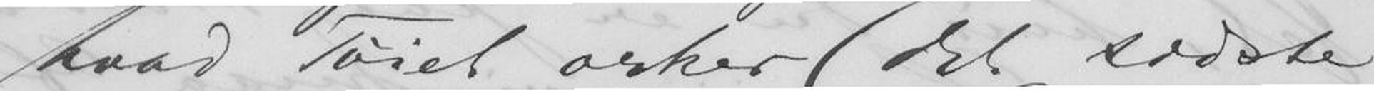hvad Tøiet orker (det sidste
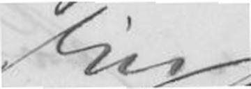Din
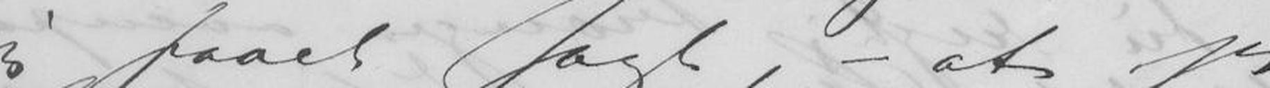jeg faaet sagt, - at jeg
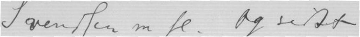Svendsen m. fl. Og sidst
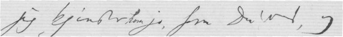jeg kjender han jo, som Du ved, og
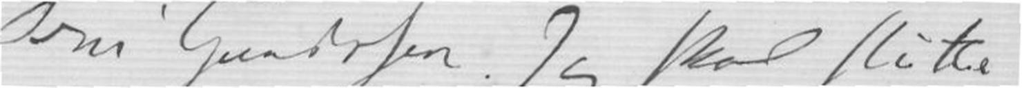Fru Gundersen. Jeg skal slutte-
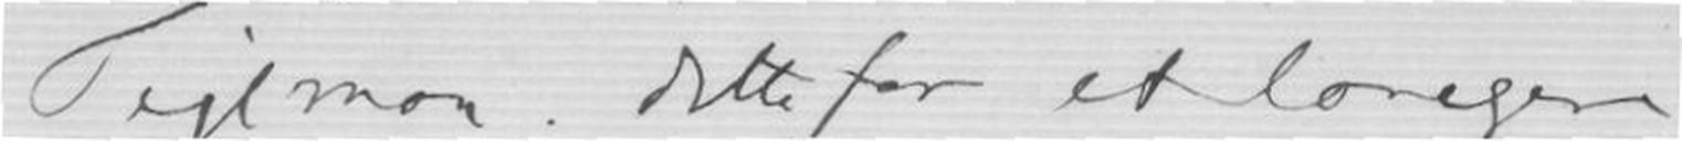Tejlman. Dette for et længere
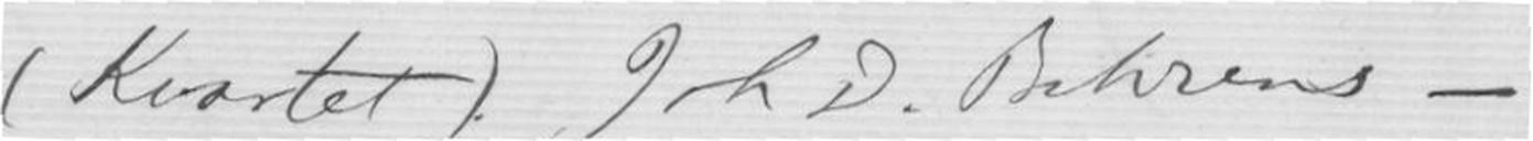(Kvartet) Joh D. Behrens -
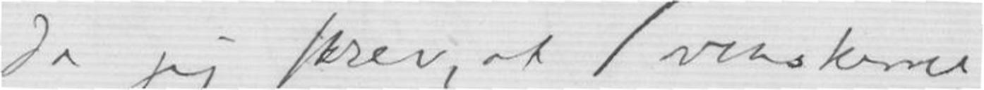da jeg skrev, at Svenskerne
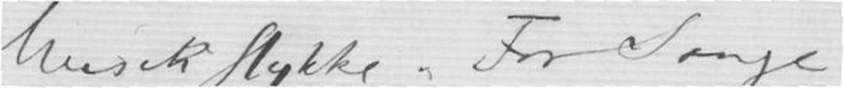Musikstykke. For Sange
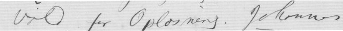vold, for Oplæsning. Johannes
